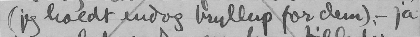(jeg holdt endog bryllup for dem), - ja
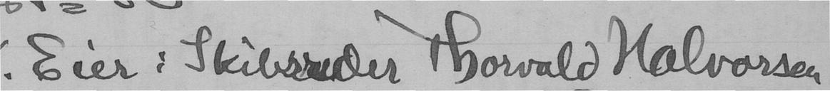Eier: Skibsreder Thorvald Halvorsen
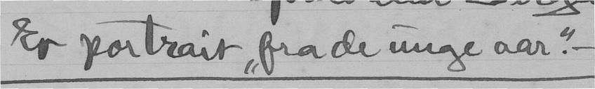Er portrait "fra de unge aar". -
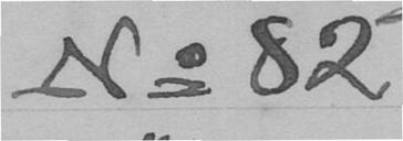No 82
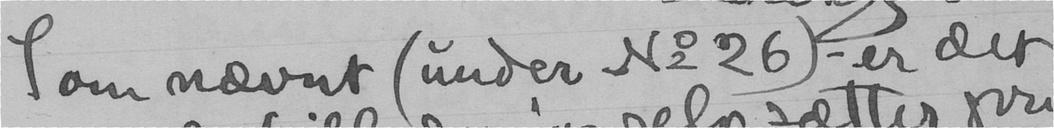Som nævnt (under No 20) er det
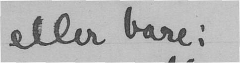eller bare:
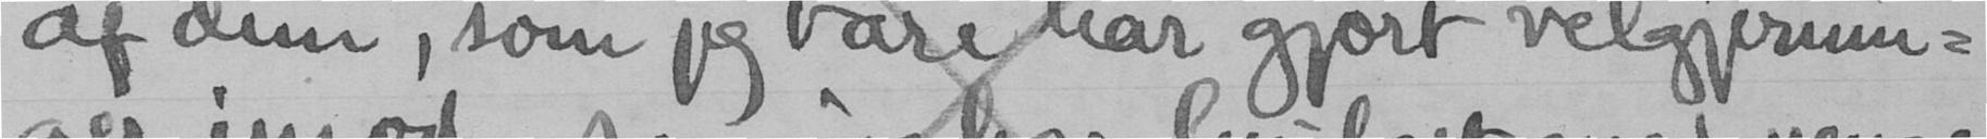af dem, som jeg bare har gjort velgjernin-
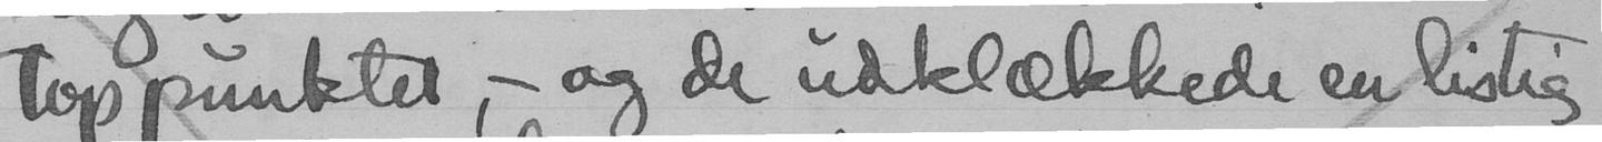toppunktet, - og de udklækkede en listig
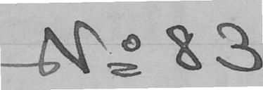No 83
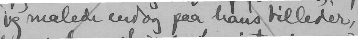jeg malede endog paa hans billeder,
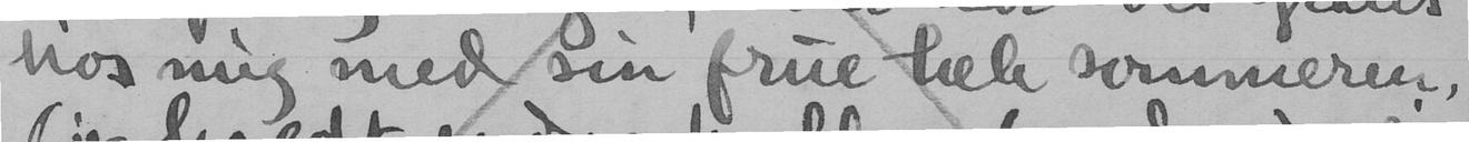hos mig med sin frue hele sommeren,
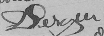Bergen
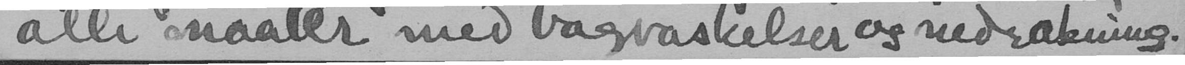alle maater med bagvaskelser og nedrakning.
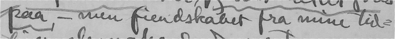paa, - men fiendskabet fra mine tid-
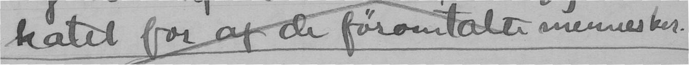hatet for af de føromtalte mennesker.
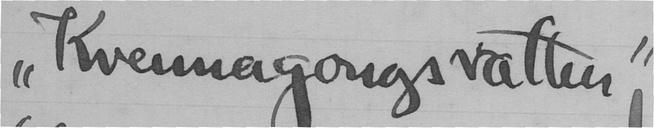"Kvemagongsvatten"
